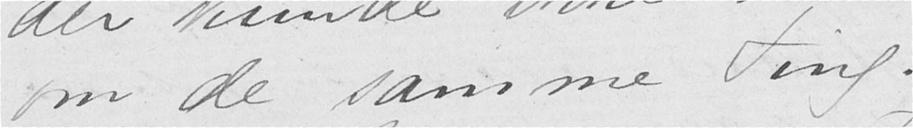om de samme Ting.
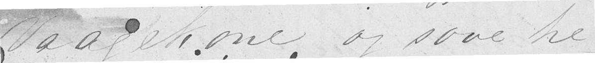Vaagekone og sove he-
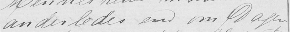anderledes end om Dagen
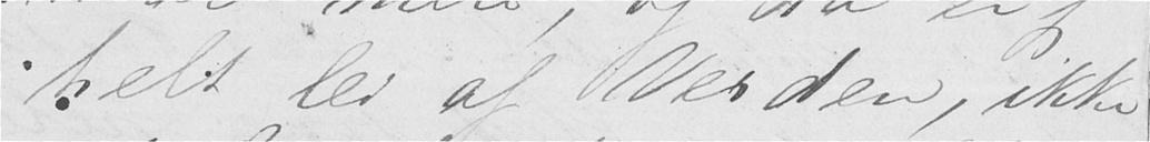helt lei af Verden, ikke
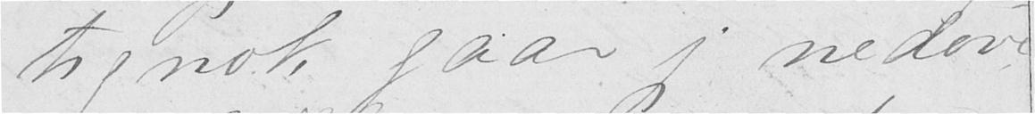tignok gaar jeg nedove
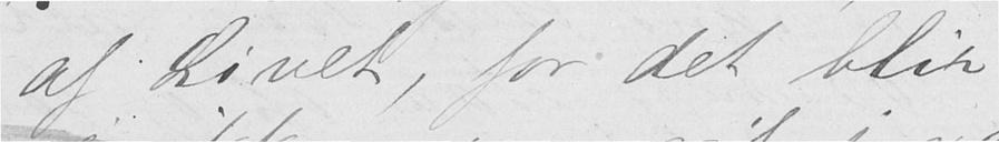af Livet, for det blir
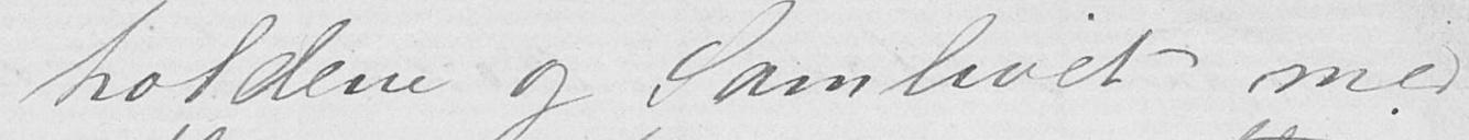holdene og Samlivet med
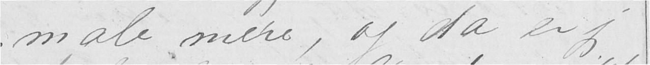male mere, og da er jeg
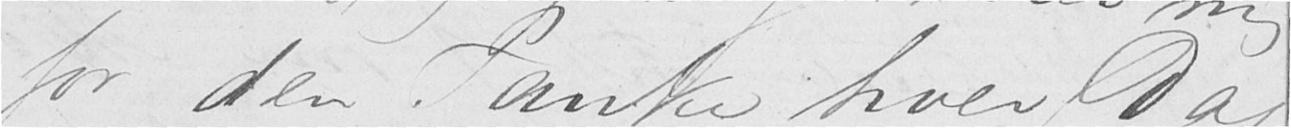for den Tanke hver Dag,
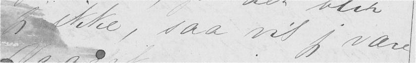jeg ikke, saa vil jeg være
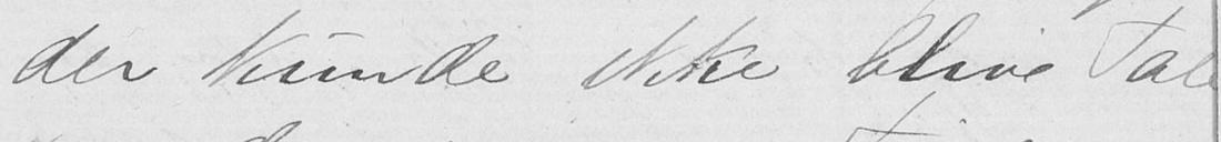der kunde ikke blive Tale
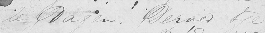le Dagen. Derved tje-
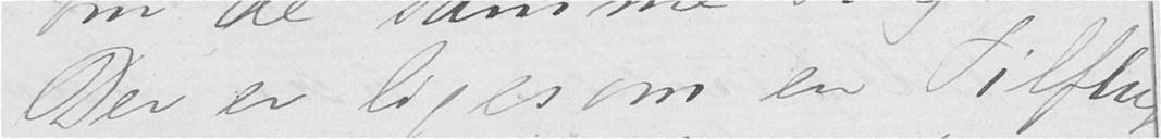Der er ligesom en Tilflug
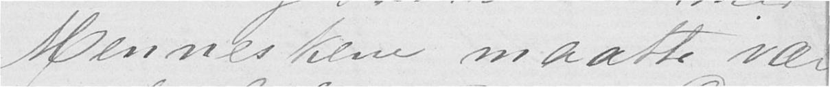Menneskene maatte være
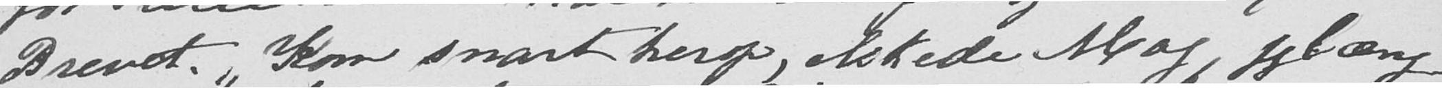Brevet. Kom snart herop, elskede Mag, jeg læng-
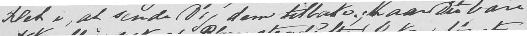Ret i at sende Dig dem tilbake. Naar Du bare
